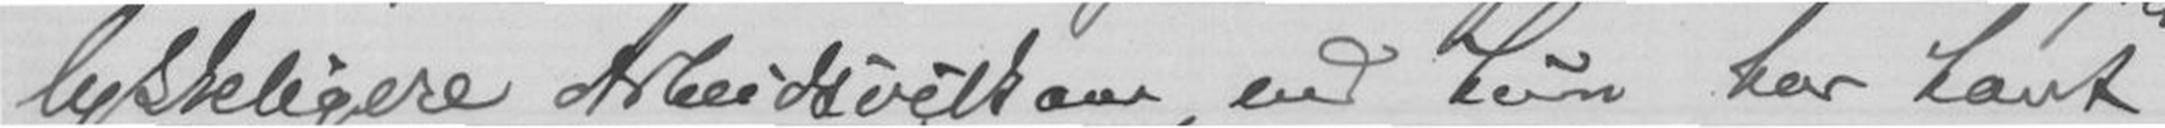lykkeligere Arbeidsvilkaar, end hun har havt
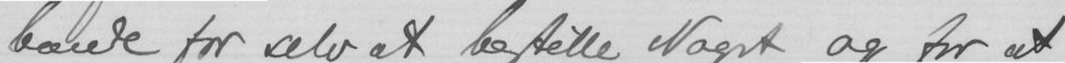baade for selv at bestille Noget og for at
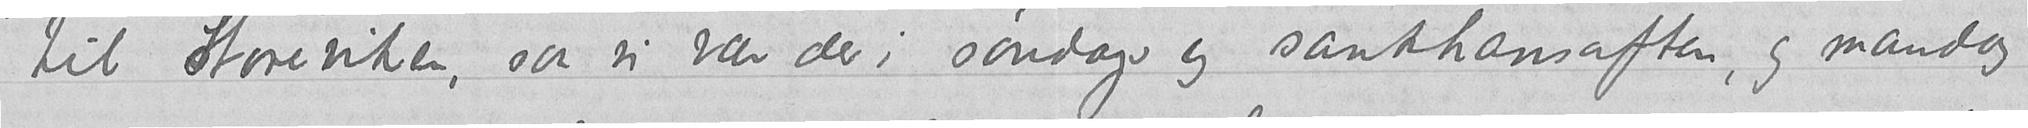til Storeviken, saa vi var der i søndags og sankhansaften, og mandag
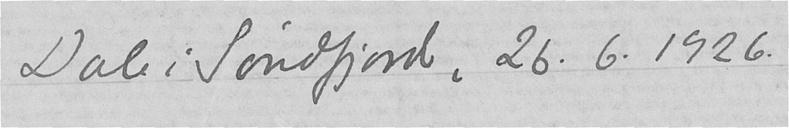Dale i Sundfjord, 26. 6. 1926.
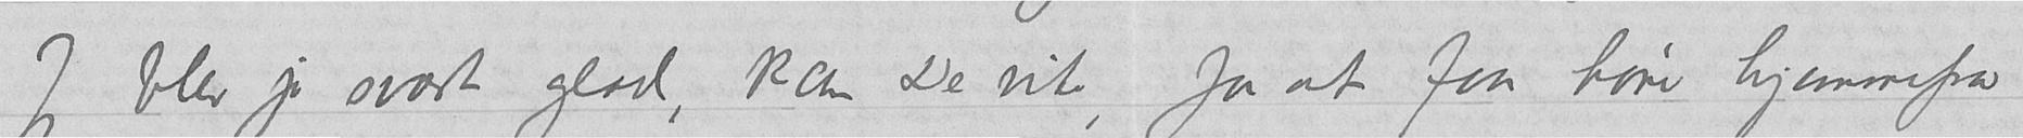Jeg blev jo svært glad, kan De vite, for at faa høre hjemmefra
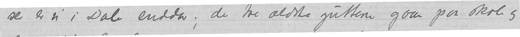ser er vi i Dale endda; de tre ældste guttene gaar paa skole og
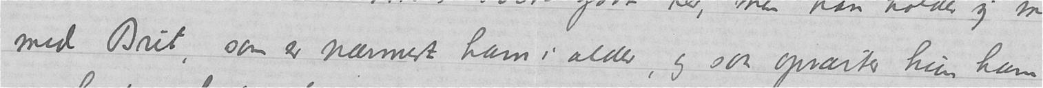med Brit, som er nærmest ham i alder, og saa opvarter hun ham
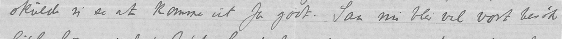skulde vi se at komme ut for godt. Saa nu blir vel vort besøk
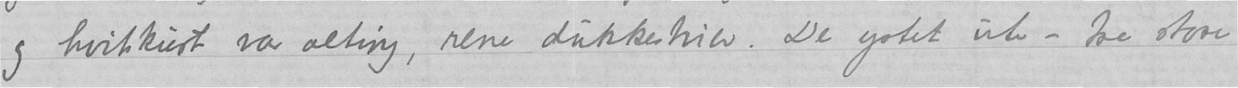og hvitskurt var alting, rene dukkestuer. De ystet ute – tre store
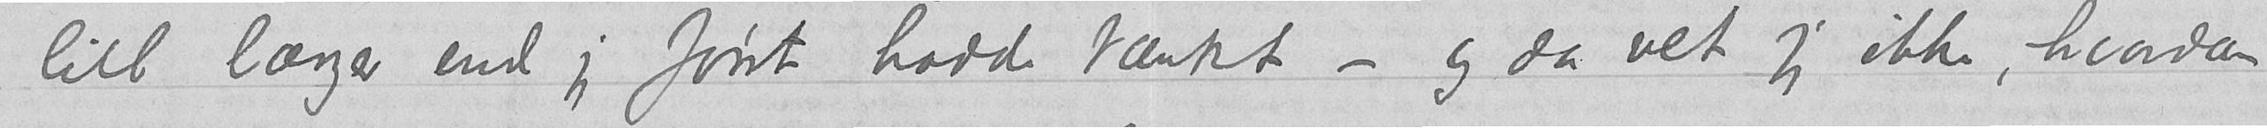litt længer end jeg først hadde tænkt – og da vet jeg ikke, hvordan
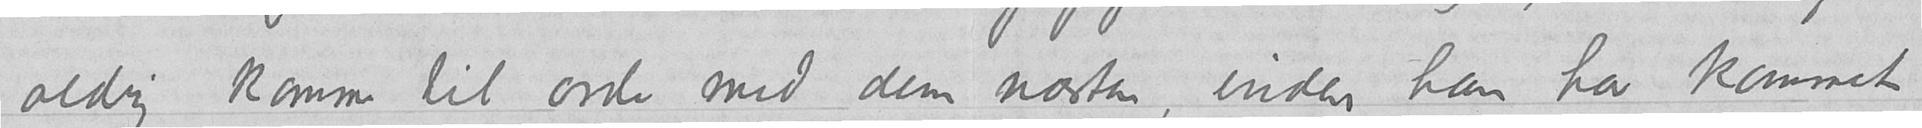aldrig komme til orde med dem næsten, inden han har kommet
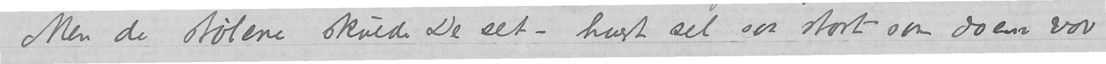Men de stølene skulde De set – hvert sel saa stort som doen vor
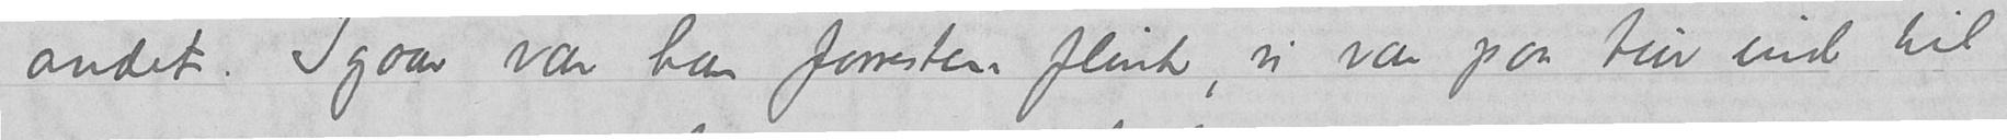andet. Igaar var han forresten flink, vi var paa tur ind til
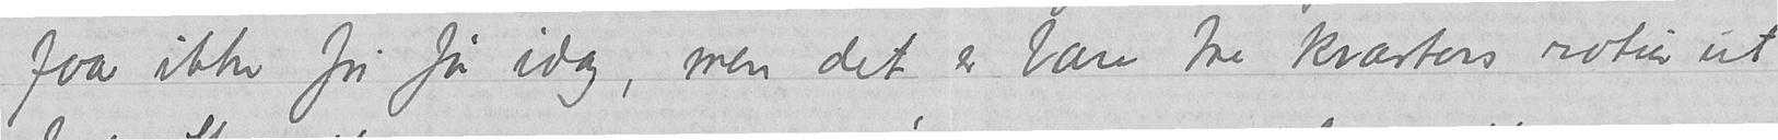faar ikke fri før idag, men det er bare tre kvarters rotur ut
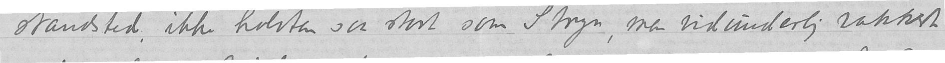strandsted, ikke halvten saa stort som Stryn, men vidunderlig vakkert
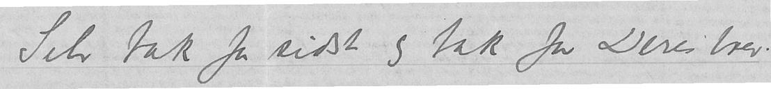Selv tak for sidst og tak for Deres brev.
